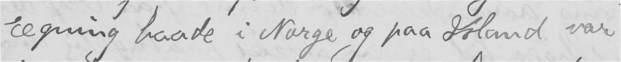regning baade i Norge og paa Island var
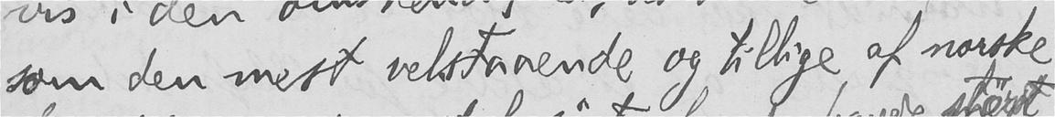som den mest velstaaende og tillige af norske
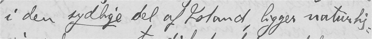i den sydlige del af Island, ligger naturlig-
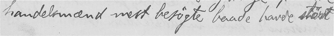handelsmænd mest besøgte baade havde størst
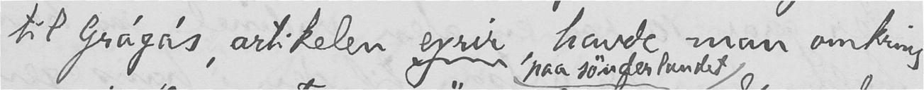til Grágás, artikelen eyrir havde man omkring
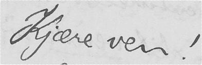Kjære ven!
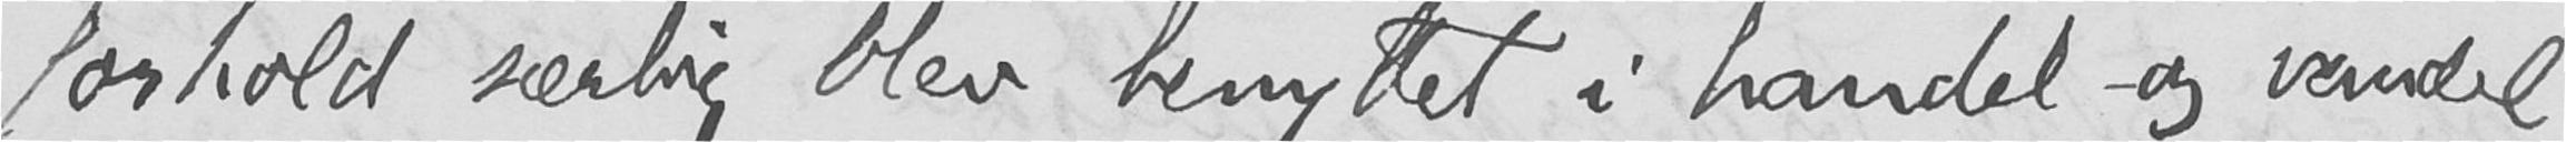forhold særlig blev benyttet i handel og vandel
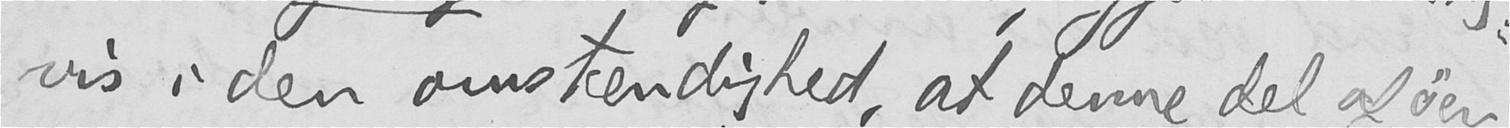vis i den omstændighed, at denne del af øen
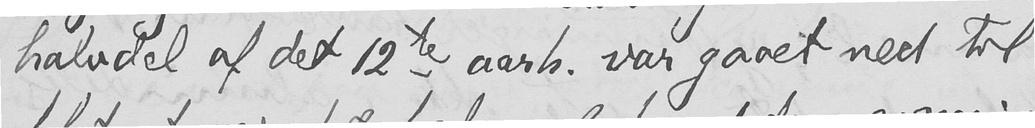halvdel af det 12te aarh. var gaaet ned til
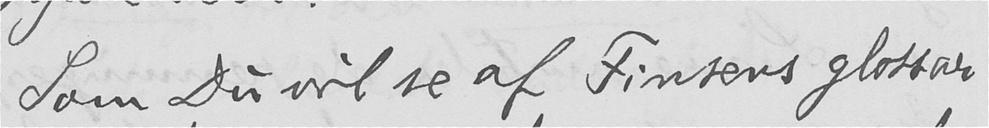Som Du vil se af Finsens glossar
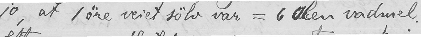jo, at 1 øre veiet sølv var = 6 alen vadmel.
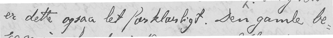er dette ogsaa let forklarligt. Den gamle be-
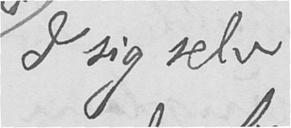I sig selv
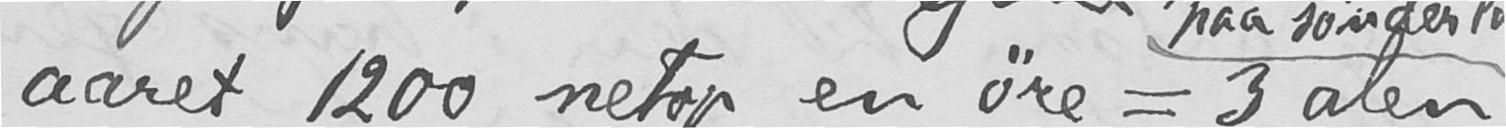aaret 1200 netop en øre = 3 alen
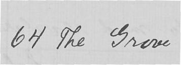64 The Grove
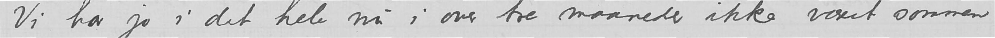Vi har jo i det hele nu i over tre maaneder ikke været sammen
